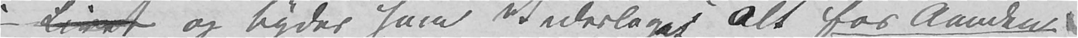i Livet og byder ham Vederlag et i Alt for Aanden
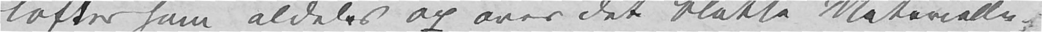løfter ham aldeles op over det blotte Materielle
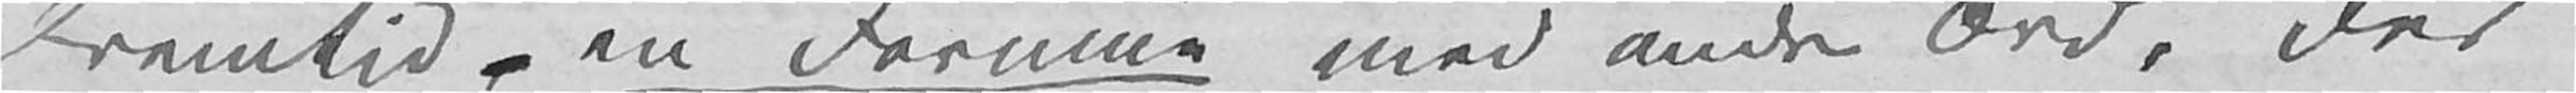Fremtid – en Formue med andre Ord, der
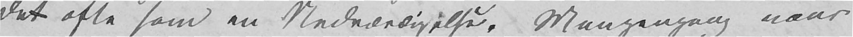det ofte som en Nedværdigelse. Mangengang naar
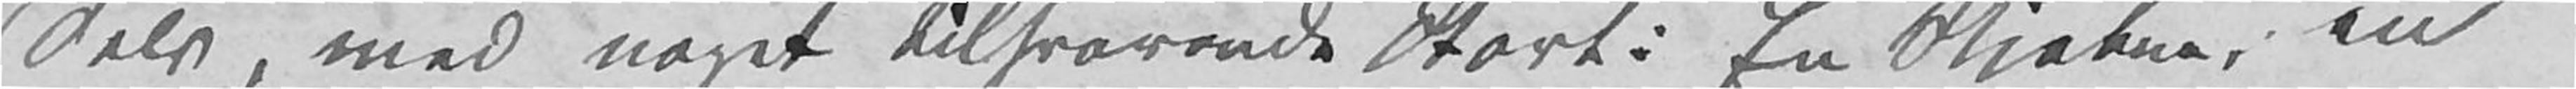Selv, med noget tilsvarende Stort: En Skjebne, en
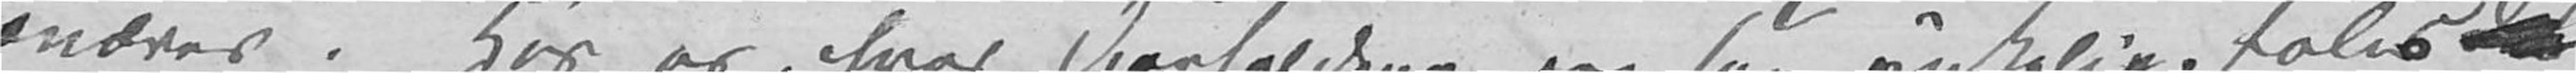ændres. Hos os, hvor Forholdene ere saa ynkelige, føles <gap /></del>
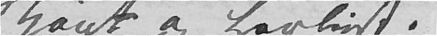Skjønt og Herligt.
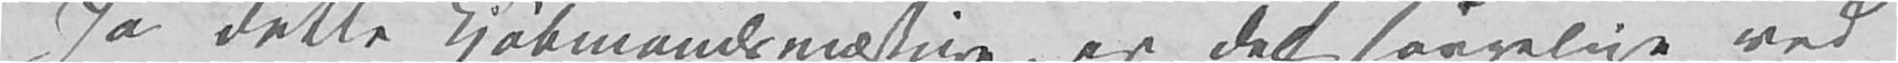Ja dette Kjøbmandsmæssige, er det sørgelige ved
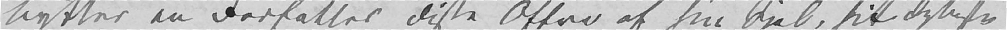bytter en Forfatter disse Offre af sin Sjel, sit dybeste
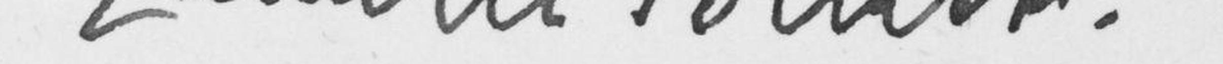Camilla Collett.
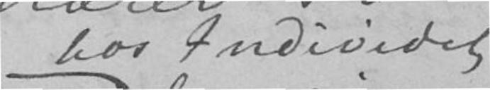hos Individet
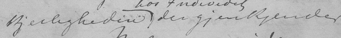kjerligheden der gjenkjender
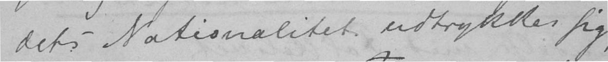dets Nationalitet udtrykke sig,
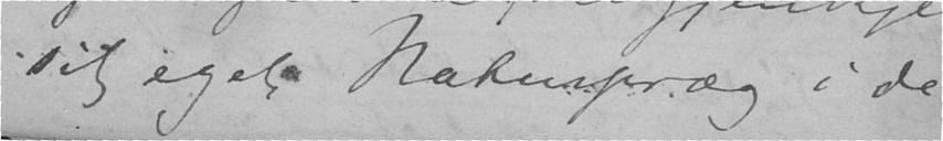sit eget Naturpræg i de
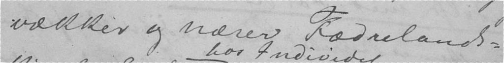vækker og nærer Fædrelands-
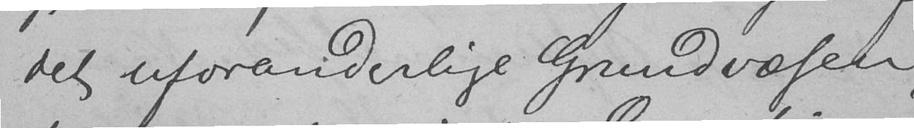det uforanderlige Grundvæsen,
